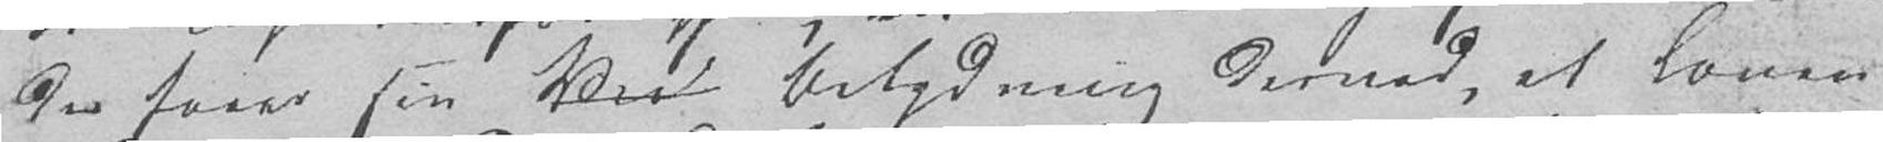der faaer sin Verd Betydning derved, at Loven
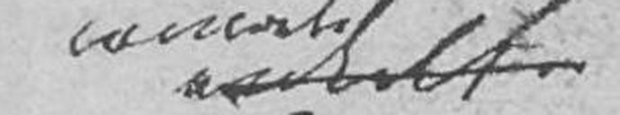enkelte
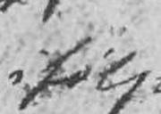ifølge
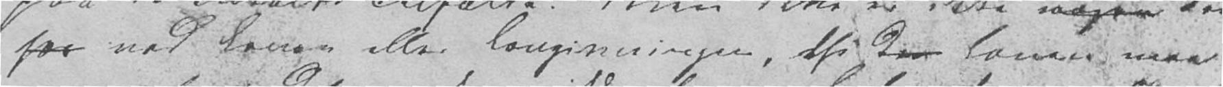for ved Loven eller Lovgivningen, thi den Loven maa
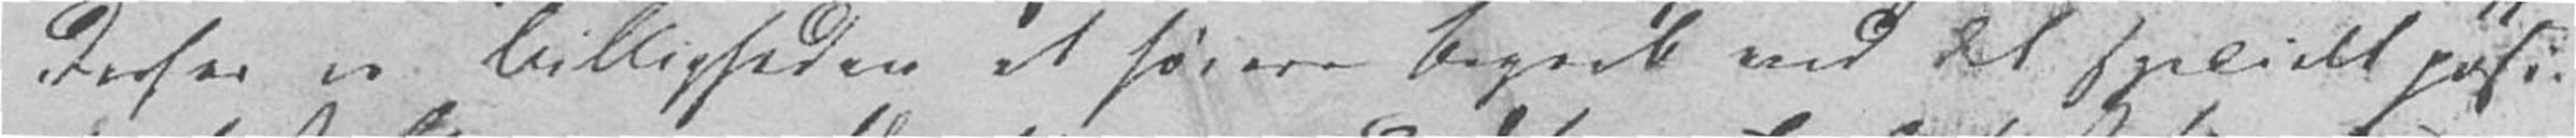Defor er Billigheden et høiere Begreb end det specielt posi-
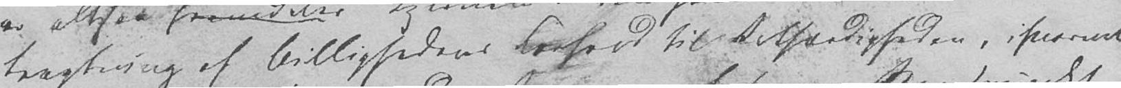tragtning af Billighedens Forhold til Retfærdigheden, ihvorvel
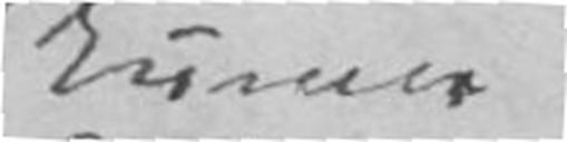kunne
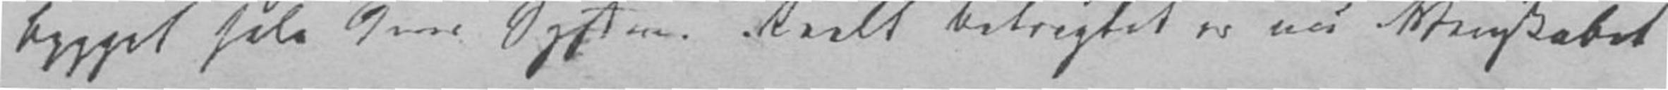bygget hele deres System. Reelt betragtet er nu Venskabet
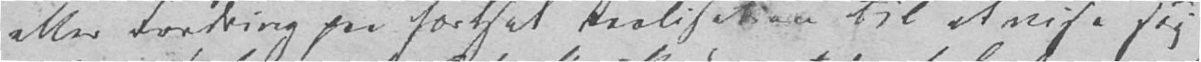eller Fordring paa fortsat Realisation til at vise sig
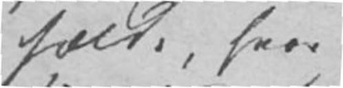fælde, hvor
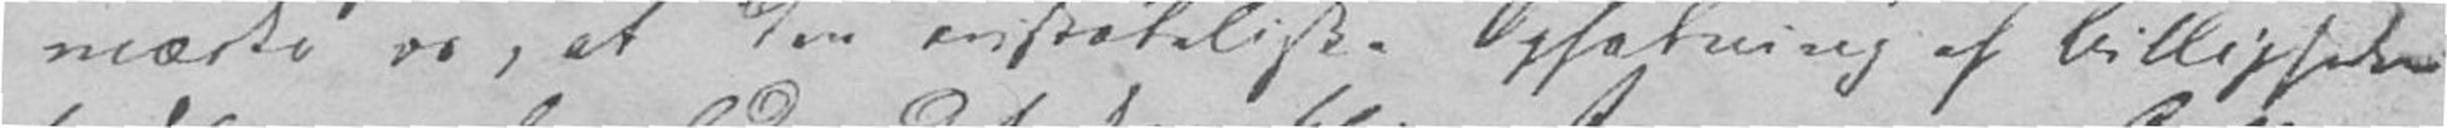mærke os, at den aristoteliske Opfatning af Billigheden
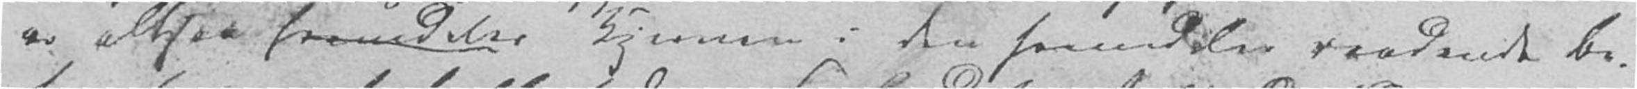er altsaa fremdeles Kjernen i den fremdeles raadende Be-
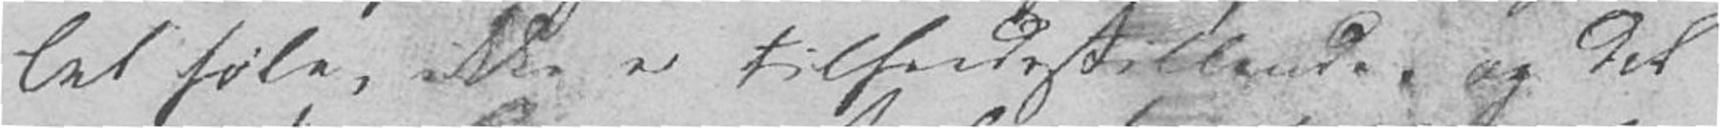let føle, ikke er tilfredsstillende, og det
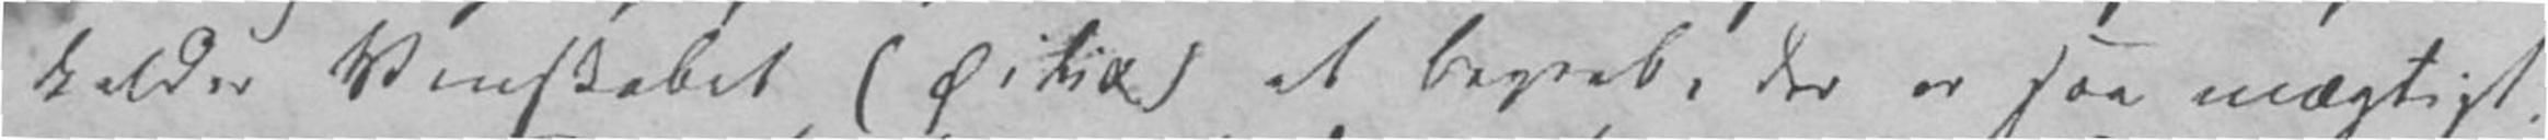kalder Venskabet (gresk) et Begreb, der er saa mægtigt,
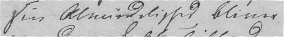sin Almindelighed bliver
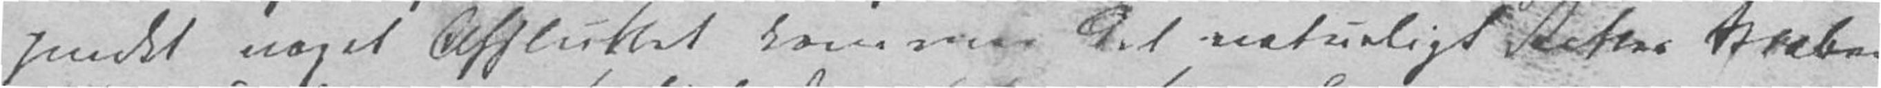punkt noget Afsluttet, kommer det naturligt Rettes Stræben
